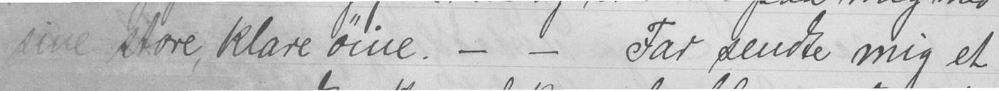sine store, klare øine. - - Far sendte mig et
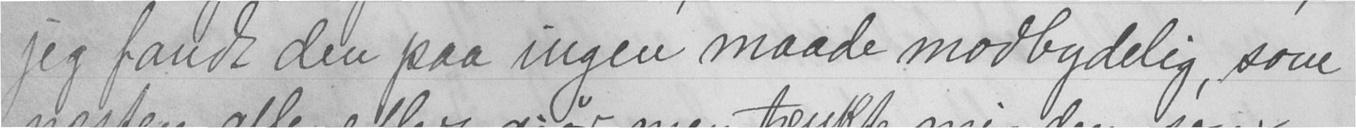jeg fandt den paa ingen maade modbydelig, som
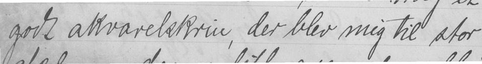godt akvarelskrin, der blev mig til stor
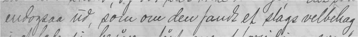endogsaa ud, som om den fandt et slags velbehag
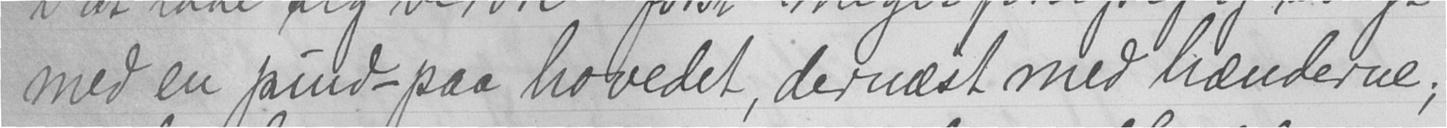med en pund- paa hovedet, dernæst med hænderne;
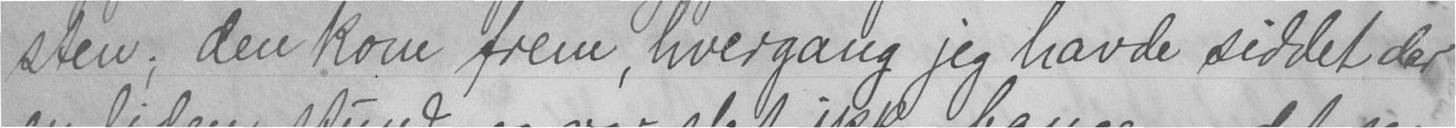sten; den kom frem, hvergang jeg havde siddet der
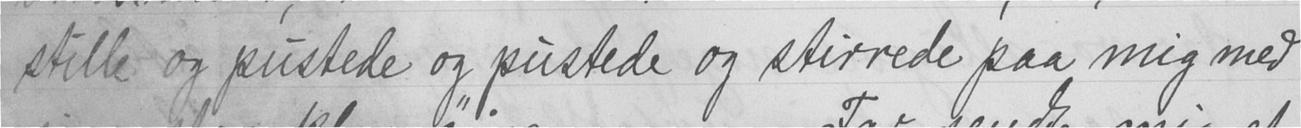stille og pustede og pustede og stirrede paa mig med
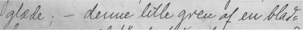glæde; - denne lille gren af en blad-
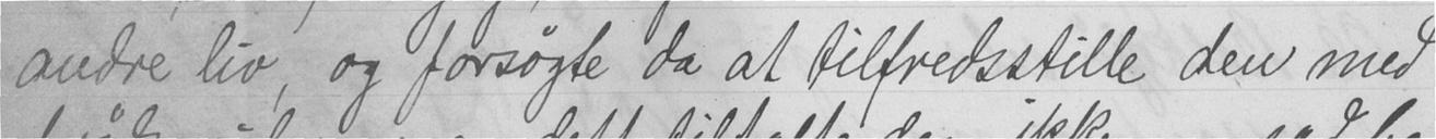andre liv, og forsøgte da at tilfredsstille den med
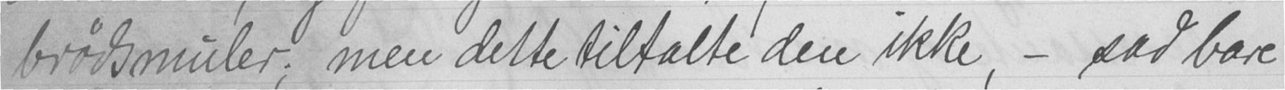brødsmuler; men dette tiltalte den ikke, - sad bare
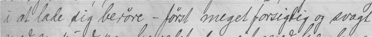i at lade sig berøre - først meget forsigtig og svagt
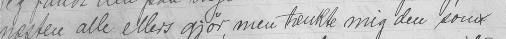næsten alle ellers gjør, men tænkte mig den som
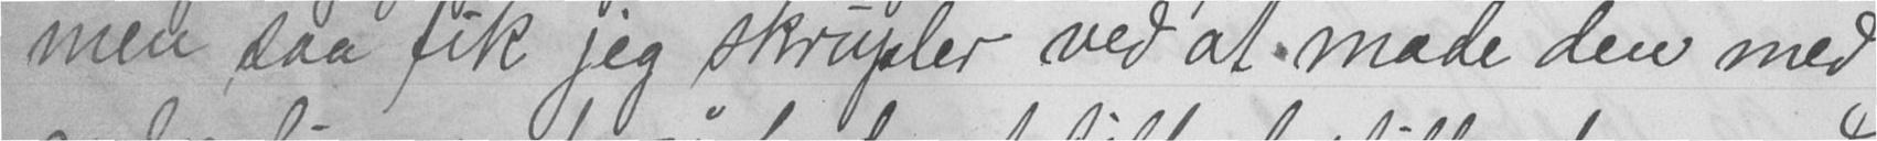men saa fik jeg skrupler ved at made den med
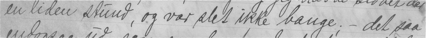en liden stund, og var slet ikke bange; - det saa
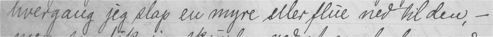hvergang jeg slap en myre eller flue ned til den, -
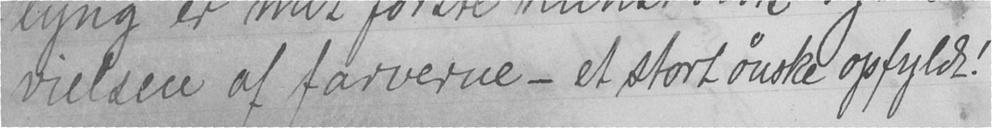vielsen af farverne - et stort ønske opfyldt!
